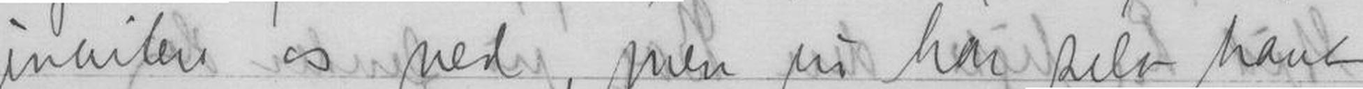invitere os ned, men vi har selv havt
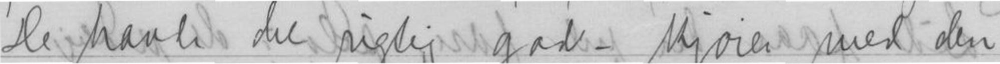De havde det rigtig godt. Kjører med den
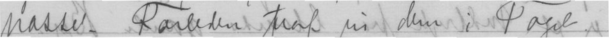passet. Forleden traf vi dem i Toget.
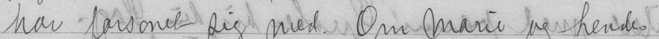har forsonet sig med. Om Marie og hendes
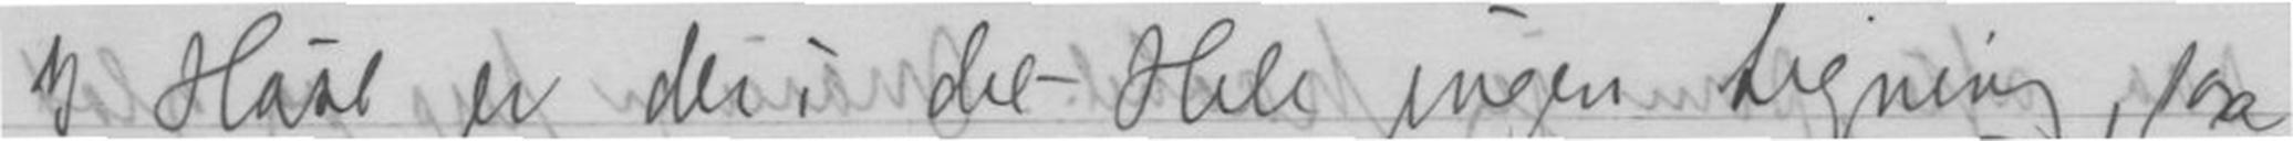I Høst er der i det Hele ingen Ligning, saa
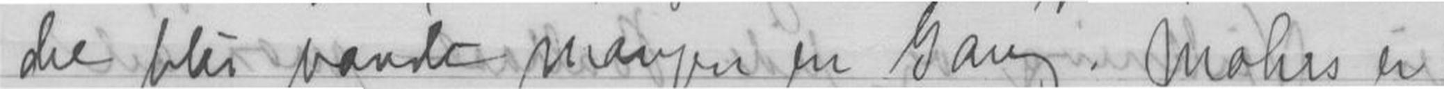det blir vondt mangen en Gang. Mohrs er
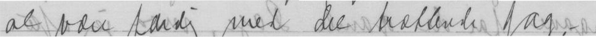at være færdig med det trættende Jag,
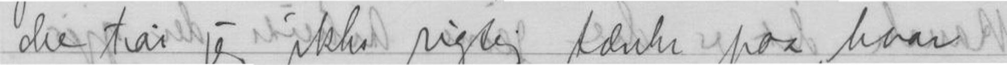det tør jeg ikke rigtig tænke paa, hvor
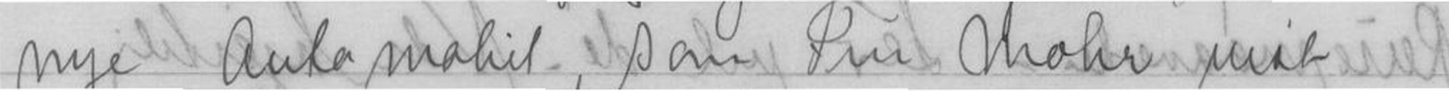nye Automobil, som Fru Mohr vist
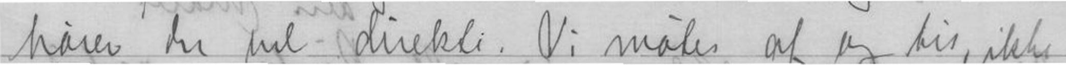hører Du vel direkte. Vi møtes af og til, ikke
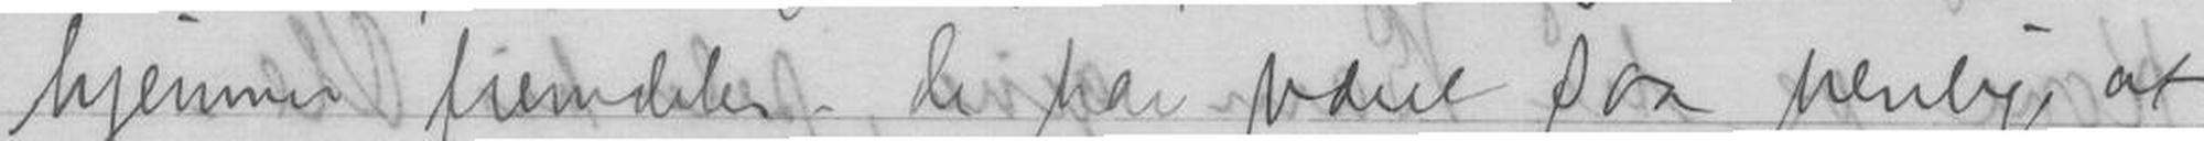hjemme fremdeles. De har været saa venlige at
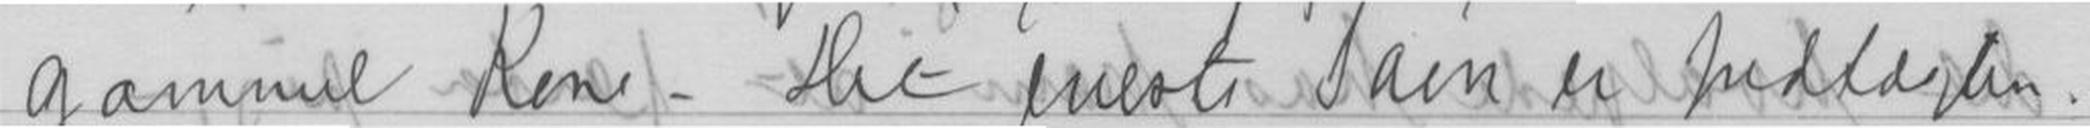gammel Kone. Det eneste Savn er Indtægten,
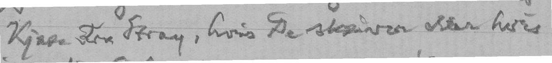Kjære Fru Stray, hvis De skriver eller hvis
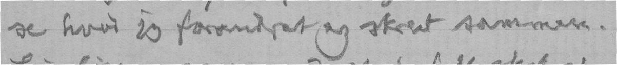se hvad jeg forandret og skrev sammen.
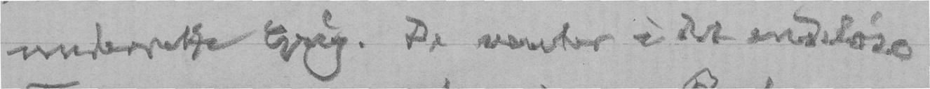underrette Grieg. De venter i det endeløse
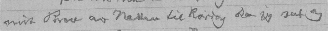mit Brev av Natten til Lørdag da jeg sat og
